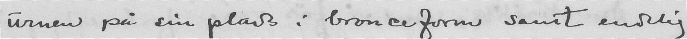urnen på sin plads i bronceform samt endelig
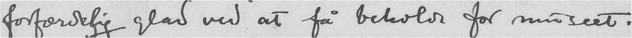forfærdelig glad ved at få beholde for museet.
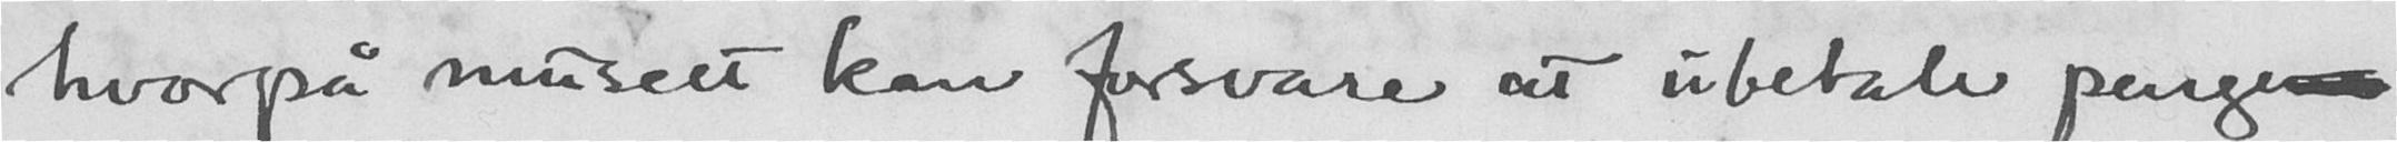hvorpå museet kan forsvare at ubetale penge
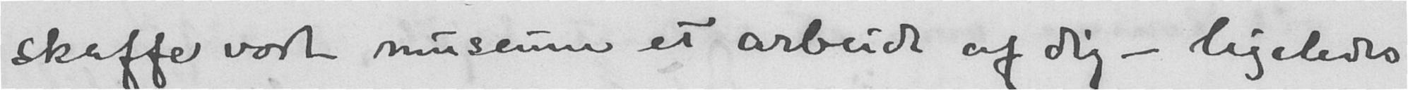skaffe vort museum et arbeide af dig - ligeledes
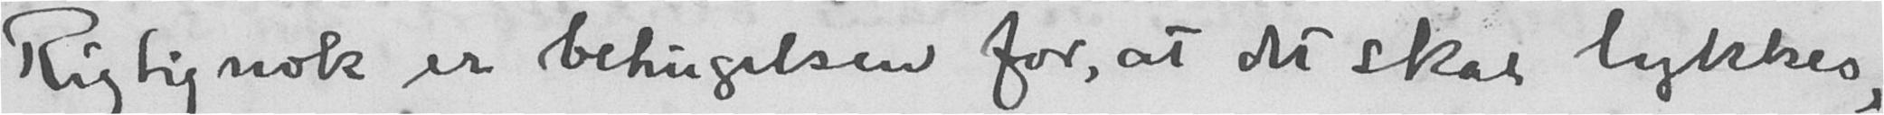Rigtignok er betingelsen for, at det skal lykkes,
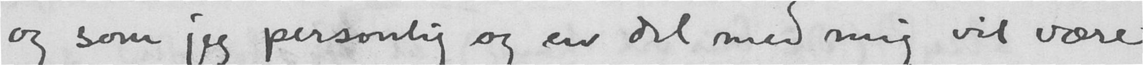og som jeg personlig og en del med mig vil være
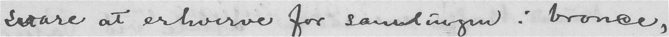svare at erhverve for samlingen i bronce,
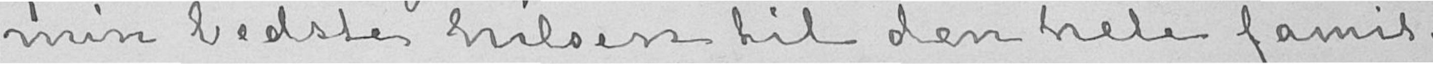min bedste hilsen til den hele famil-
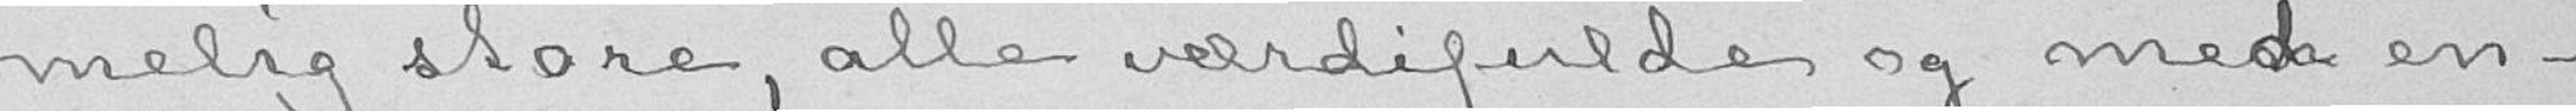melig store, alle værdifulde og med en-
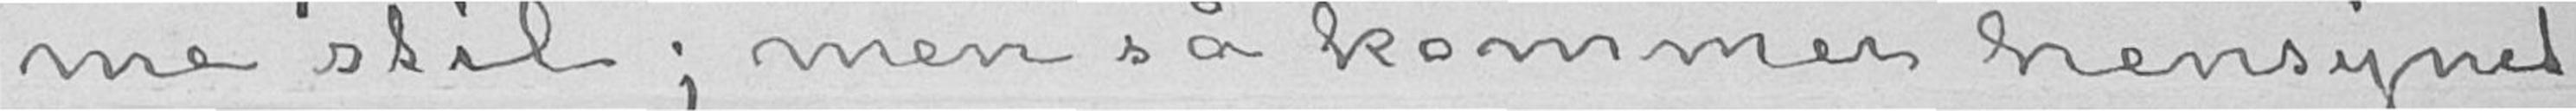me stil; men så kommer hensynet
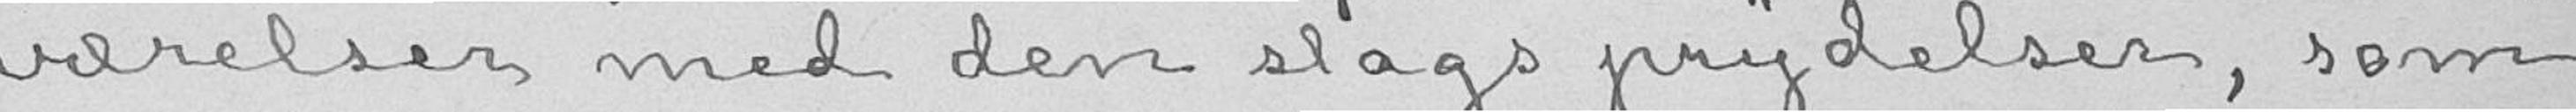værelser med den slags prydelser, som
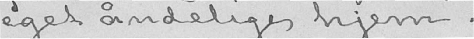eget åndelige hjem.
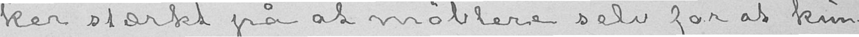ker stærkt på at møblere selv for at kun-
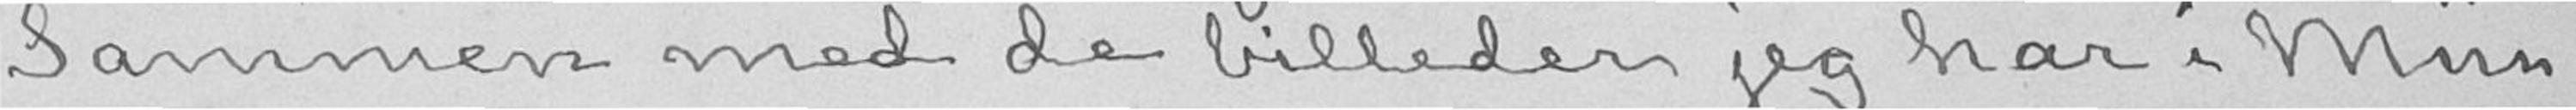Sammen med de billeder jeg har i Mün-
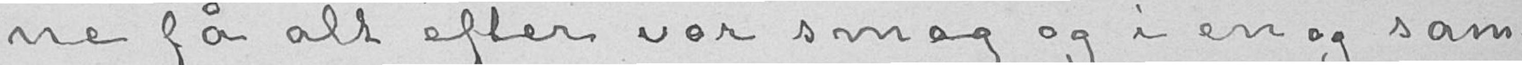ne få alt efter vor smag og i en og sam-
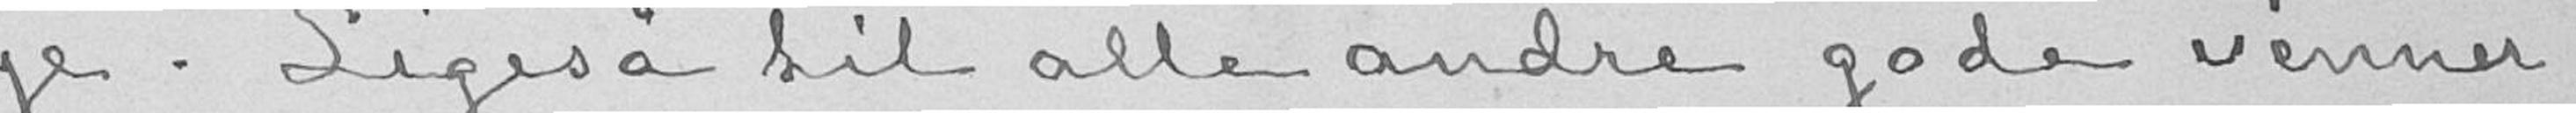je. Ligeså til alle andre gode venner.
